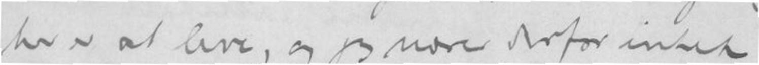her er at leve, og jeg have derfor intet
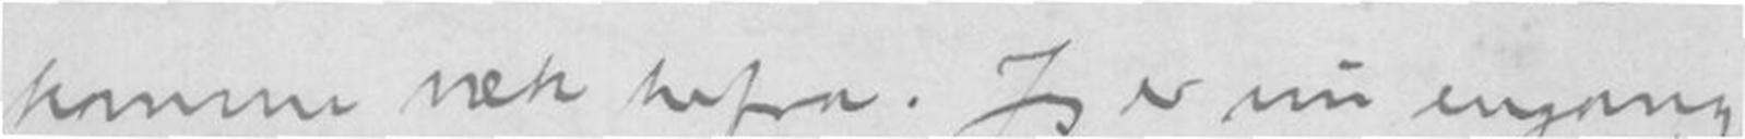komme vek herfra. Jeg er nu engang
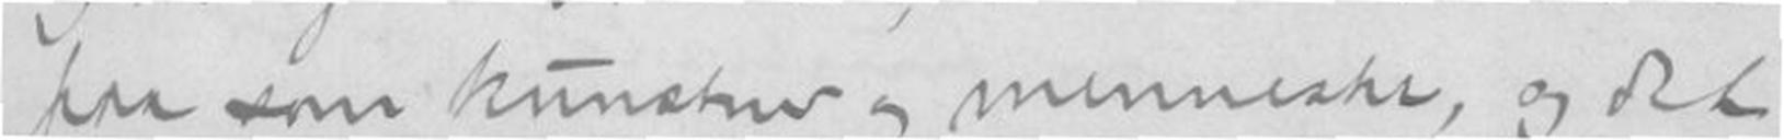paa som kunstner og menneske, og det
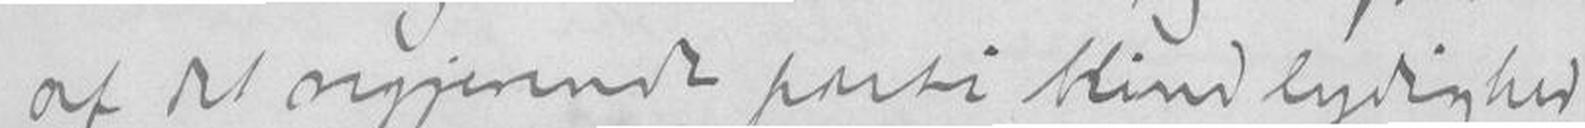af det ny regjerende parti blind lydighed
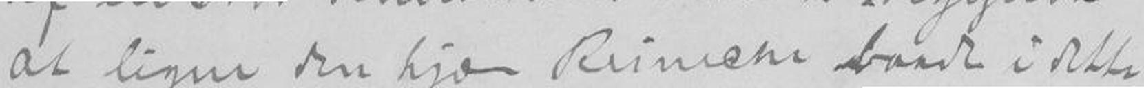at ligne kjære Reinche baade i dette
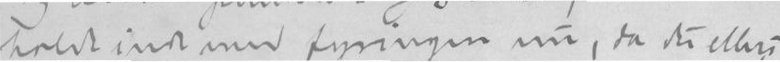holde inde med fyringen nu, da du ellers
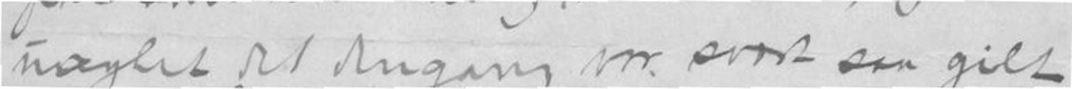uagtet det dengang var svært saa gilt
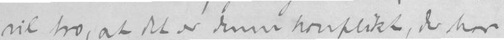vil tro, at det er denne konflikt, der har
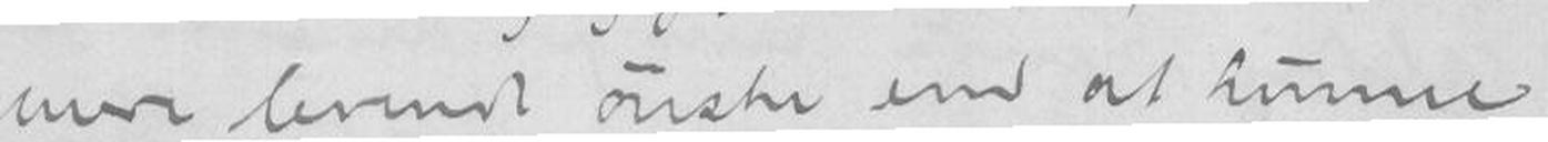mer levende ønske end at kunne
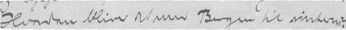Hvordan bliver det med Bergen til vinteren?
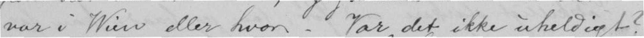var i Wien eller hvor. Var det ikke uheldigt?
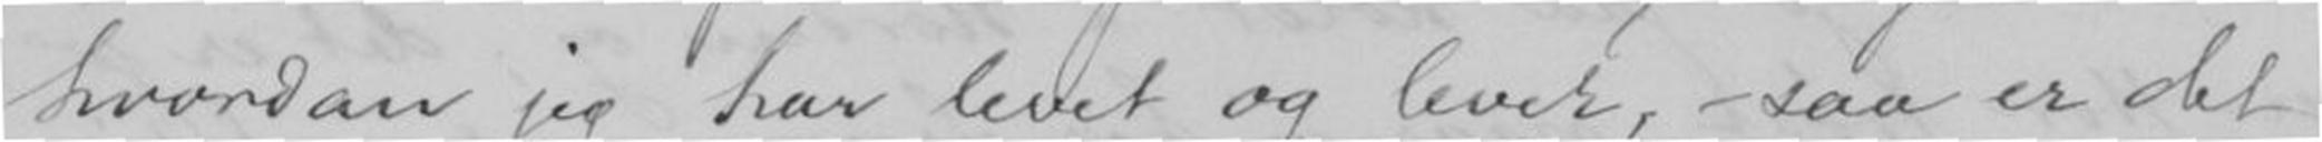hvordan jeg har levet og lever, - saa er det
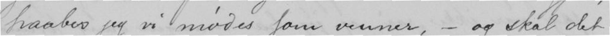haabes jeg vi mødes som venner, - og skal det
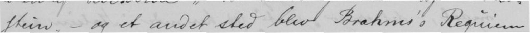stein, - og et andet sted blev Brahms's Requiem
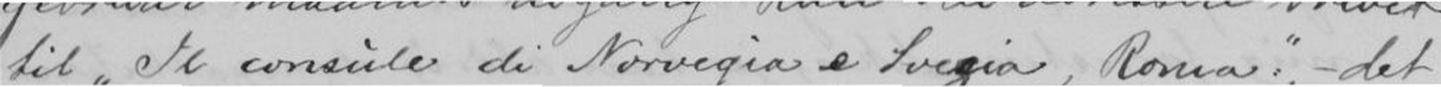til "Il consule di Norvegia & Svegia, Roma", - det
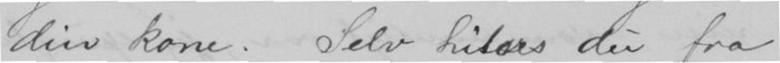din kone. Selv hilses du fra
